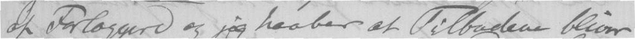af Forlæggere og jeg haaber at Tilbudene bliver
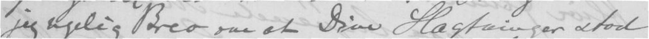jeg nylig Brev om at Dine Slægtninger stod
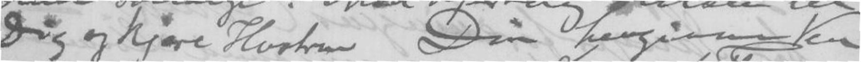Dig og kjære Hustru Din hengivne Ven
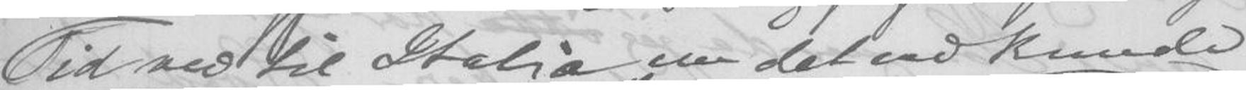Tid ned til Italia om det end kunde
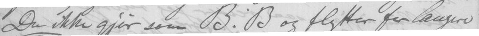Du ikke gjør som B. B. og flytter for længere
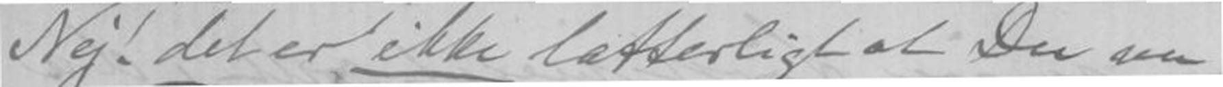Nej! det er ikke latterligt at Du nu
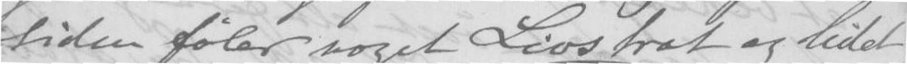tiden føler noget Livstræt og lidet
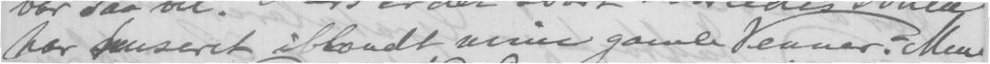har huseret iblandt mine gamle Venner. Men
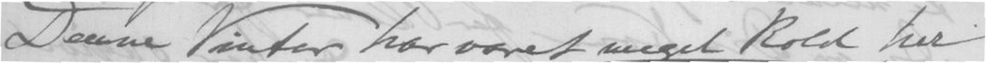Denne Vinter har været meget kold her
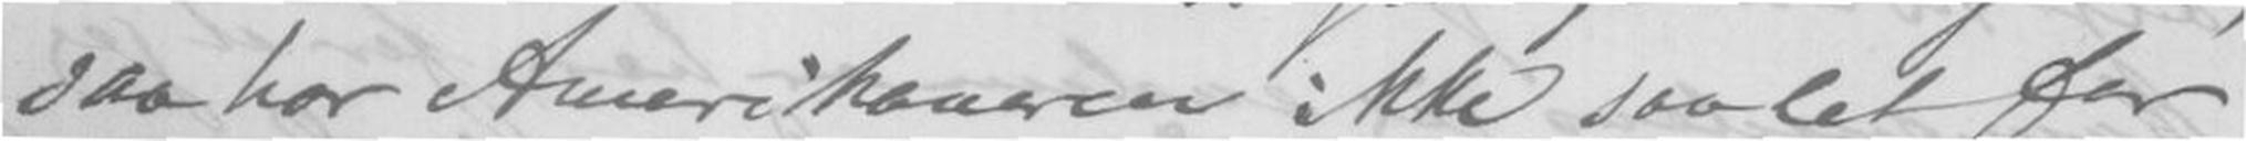saa har Amerikaneren ikke saa let for
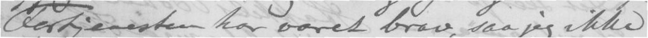Fortjenesten har været brav, saa jeg ikke
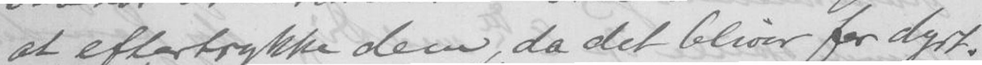at eftertrykke dem, da det bliver for dyrt.
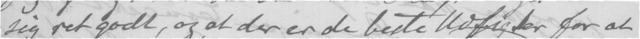sig ret godt, og at der er de bedste Udsigter for at
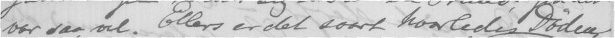var saa vel. Ellers er det svært hvorledes Døden
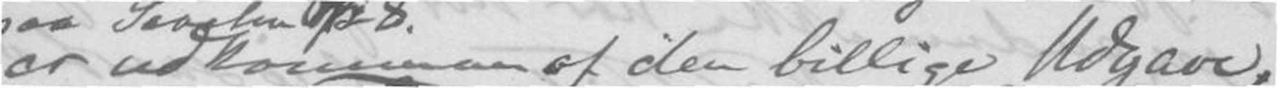er udkommen af den billige Udgave,
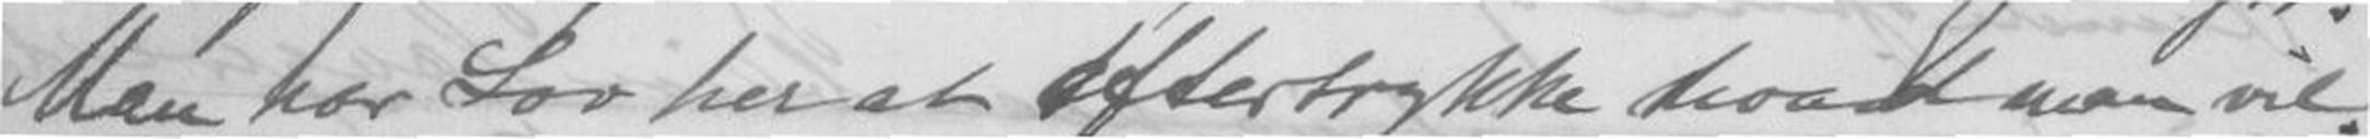Man har Lov her at eftertrykke hvad man vil.
